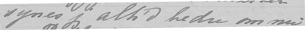synes jeg altid bedre om mi-
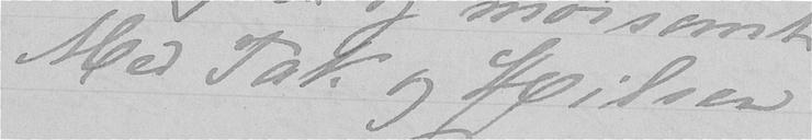Med Tak og Hilsen
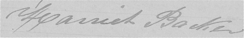Harriet Backer
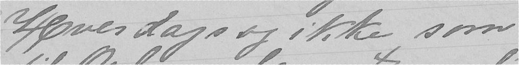Hverdags og ikke som
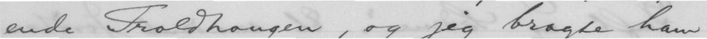ende Troldhaugen, og jeg bragte ham
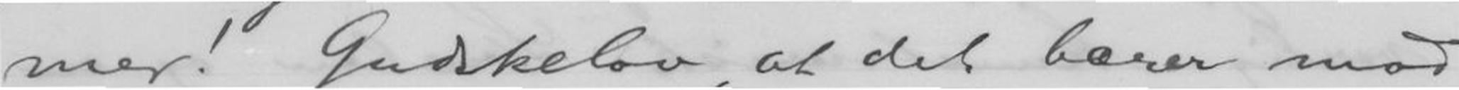mer! Gudskelov, at det bærer mod
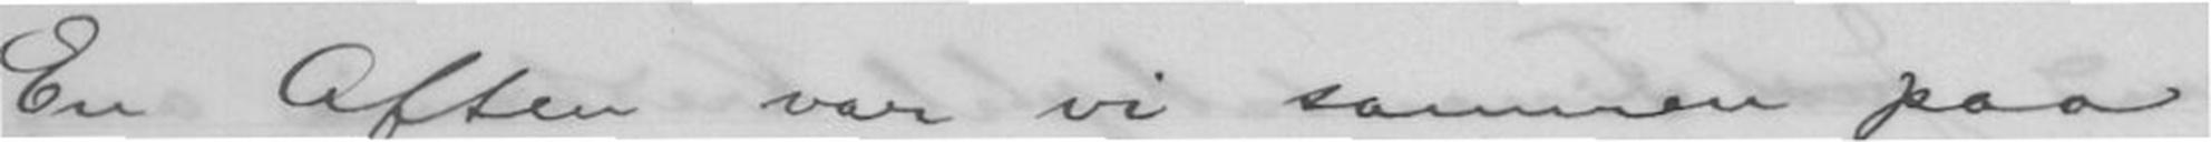En Aften var vi sammen paa
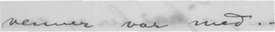venner var med.
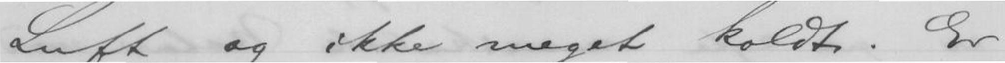Luft og ikke meget koldt. Er
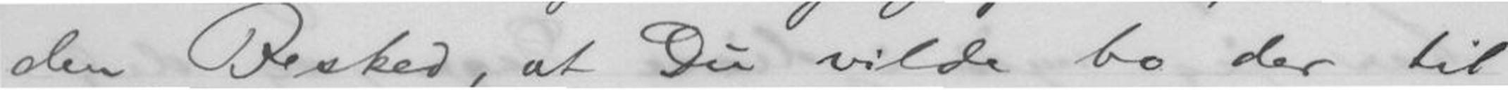den Besked, at Du vilde bo der til
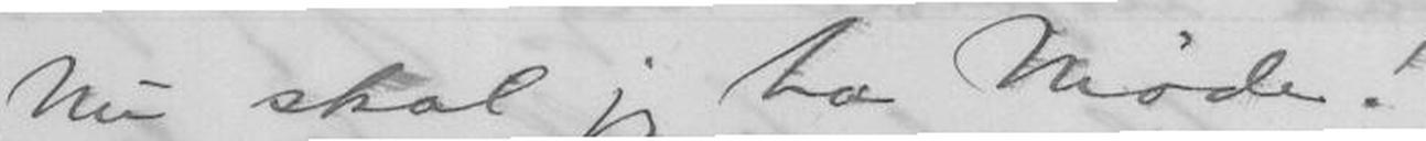Nu skal jeg ha Møde!
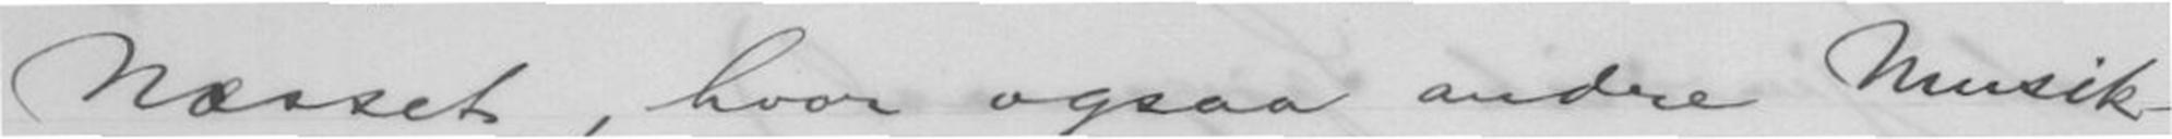Næsset, hvor ogsaa andre Musik-
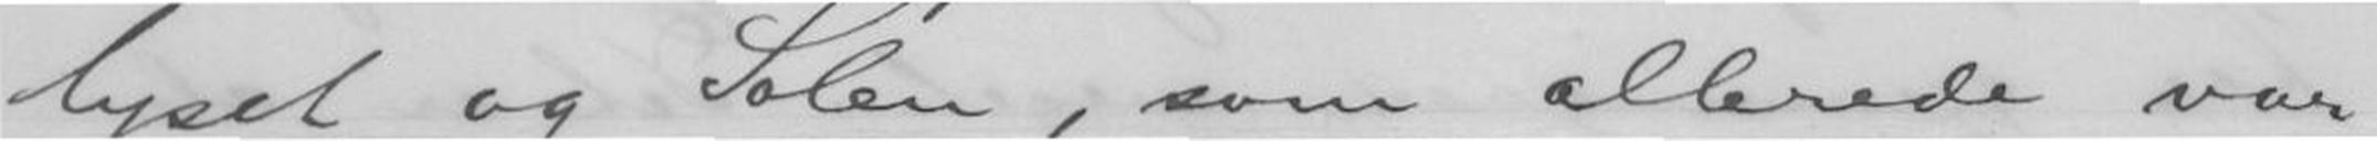lyset og Solen, som allerede var-
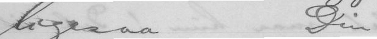ligesaa Din
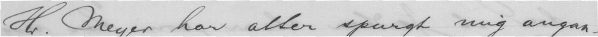Hr. Meyer har atter spurgt mig angaa-
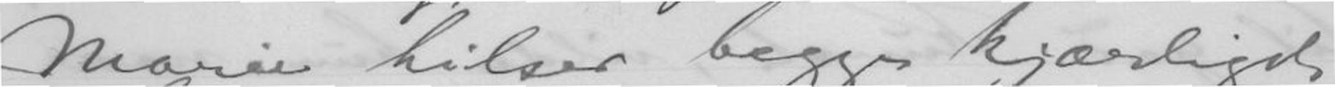hilser begge kjærligst,
